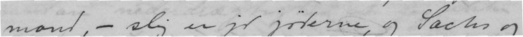mand, - slig er jo jøderne, og Sachs og
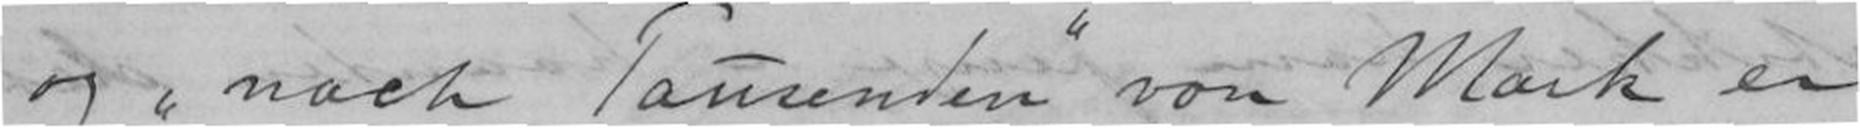og "nach Tausenden" von Mark er
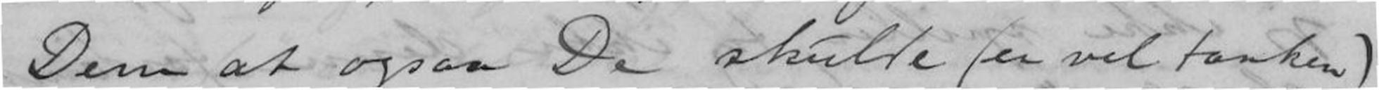Dem, at ogsaa De skulde (er vel tanken)
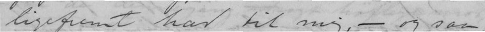ligefremt had til mig, - og saa
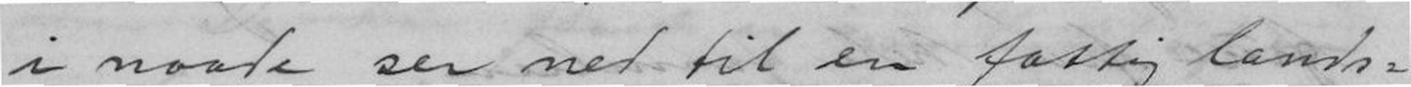i naade ser ned til en fattig lands-
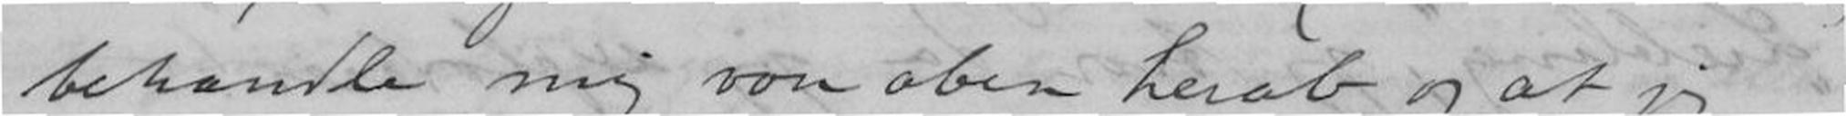behandle mig von oben herab og at jeg
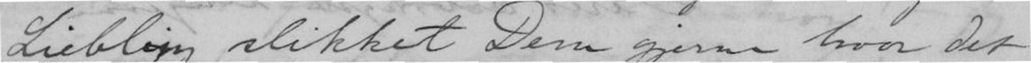Liebling slikket Dem gjerne hvor det
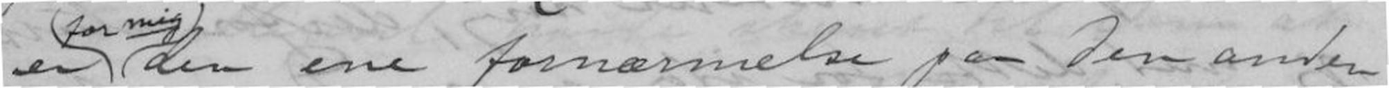er den ene fornærmelse paa den anden,
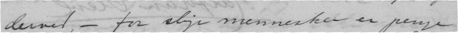derved, - for slige mennesker er penge
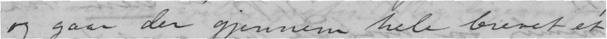og gaar der gjennem hele brevet et
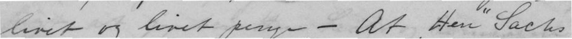livet og livet penge. - At "Herr" Sachs
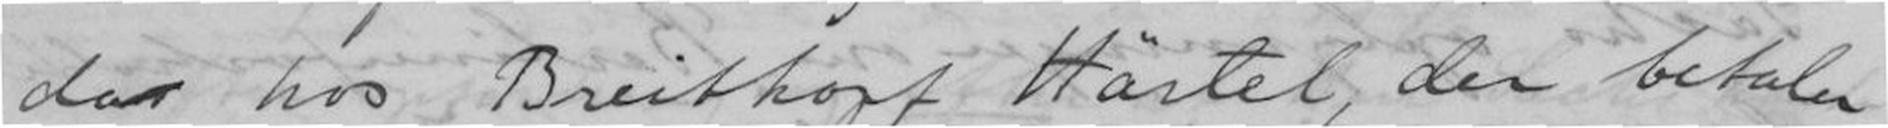da hos Breitkopf Härtel, der betaler
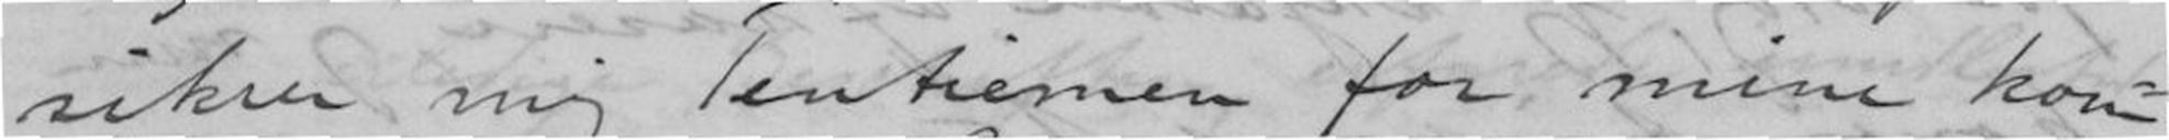sikrer mig Tentiemen for mine kom-
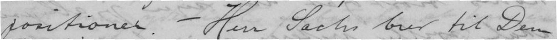positioner. - Herr Sachs brev til Dem
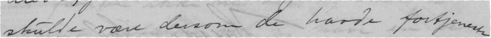skulde være dersom de havde fortjeneste
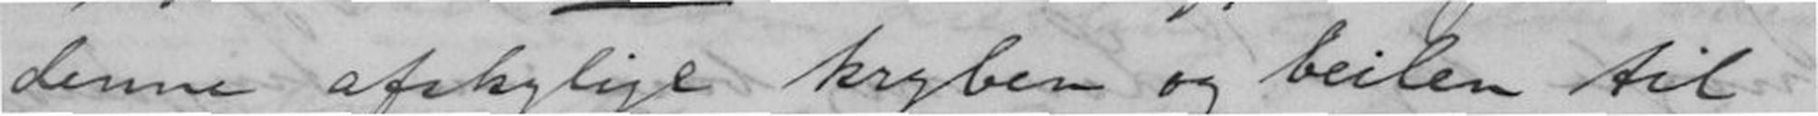denne afskylige kryben og beilen til
